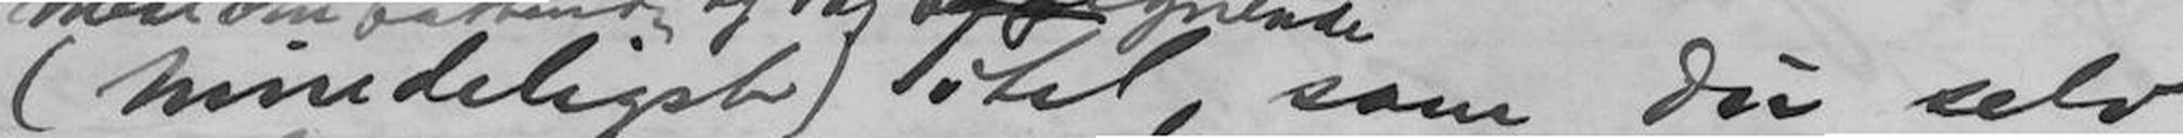mindeligste) Titel, om du selv
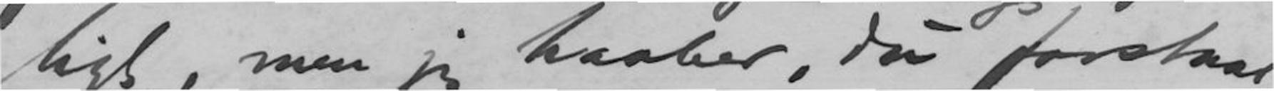ligt, men jeg haaber, du forstaar
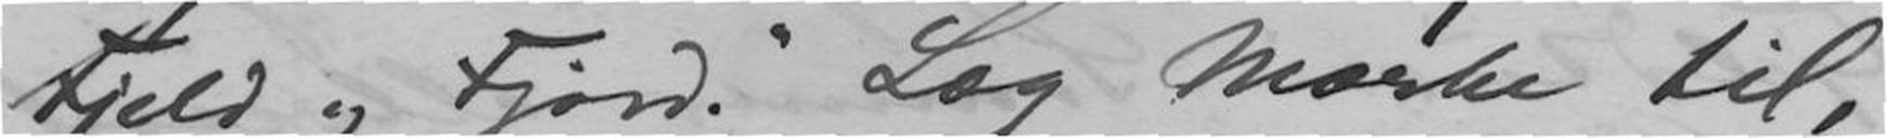Fjeld og Fjord". Læg Mærke til,
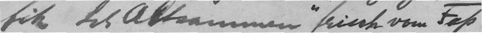fik det Altsammen "frisch vom Fass",
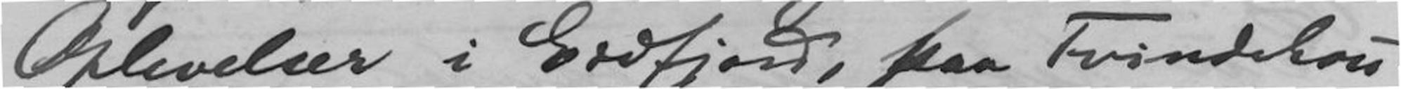Oplevelser i Eidfjord, paa Tvindehou-
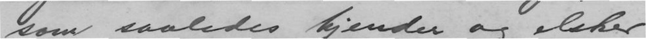som saaledes kjender og elsker
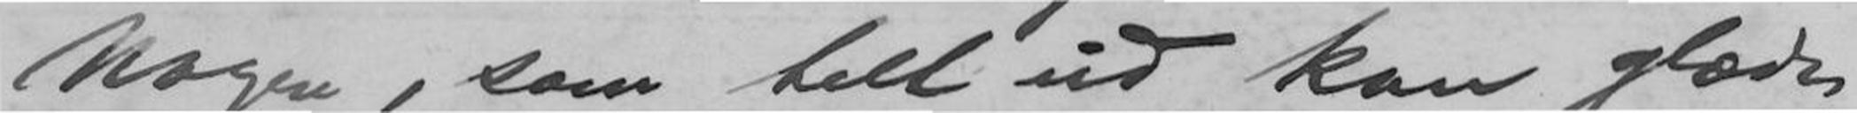Nogen, som helt ud kan glæde
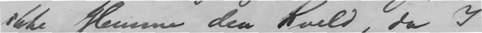ikke glemme den Kveld, da I
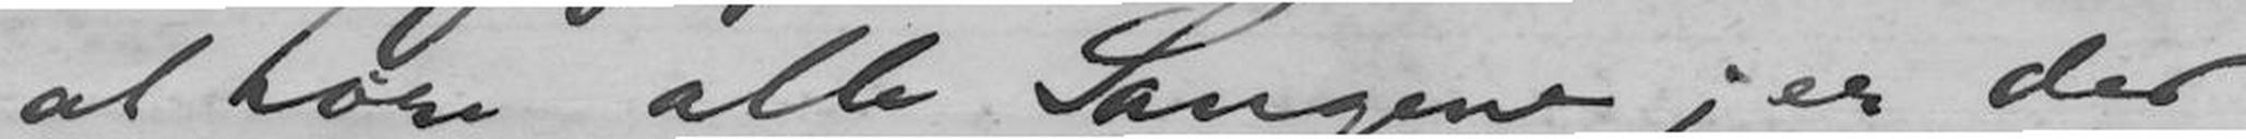at høre alle Sangerne; er der
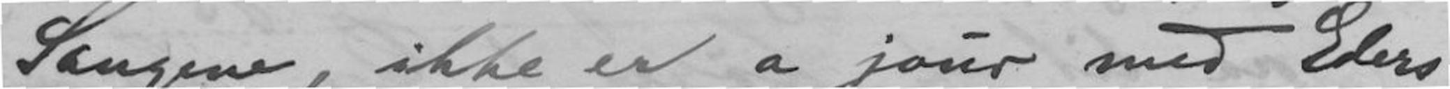Sanger, ikke er a jour med Eders
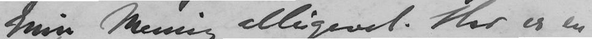min Mening alligevel. Her er en
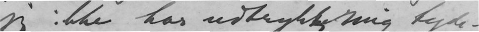jeg ikke har udtrykket mig tyde-
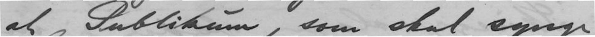at Publikum, som skal synge
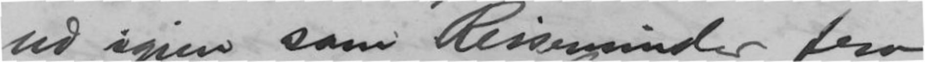ud igjen " Reiseminder fra
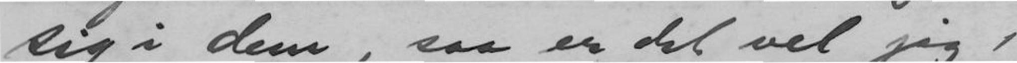sig i den, saa er det vel jeg,
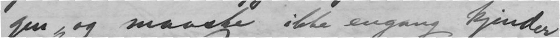gen, og maaske ikke engang kjender
